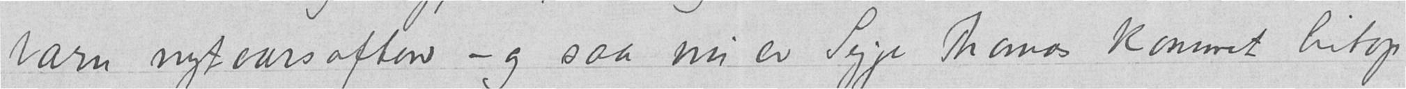barn nytaarsaften - og saa nu er Sigge Thomas kommet hitop
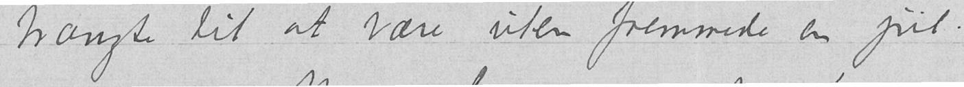trængte til at være uten fremmede en jul.
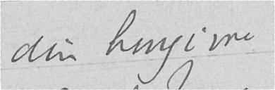din hengivne
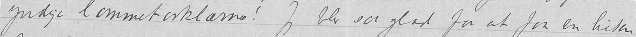yndige lommetørklærne! Jeg blev saa glad for at faa en hilsen
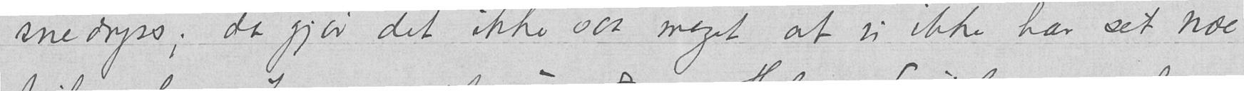snedryss; da gjør det ikke saa meget at vi ikke har set noe
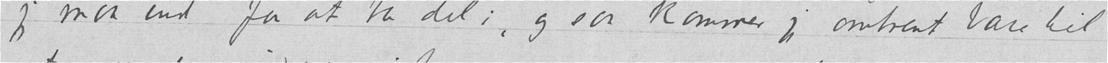jeg maa ind for at ta del i, og saa kommer jeg omtrent bare til
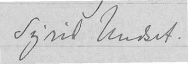Sigrid Undset.
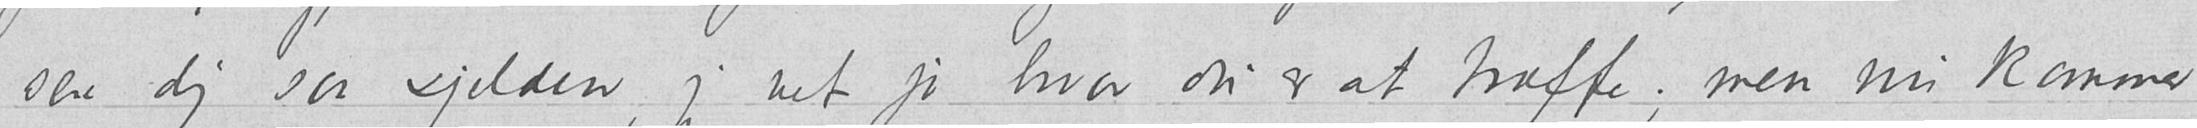ser dig saa sjelden, jeg vet jo hvor du er at træffe; men nu kommer
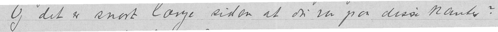Og det er svært længe siden at du var paa disse kanter?
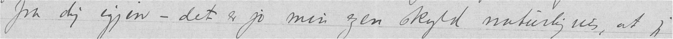fra dig igjen – det er jo min egen skyld naturligvis, at jeg
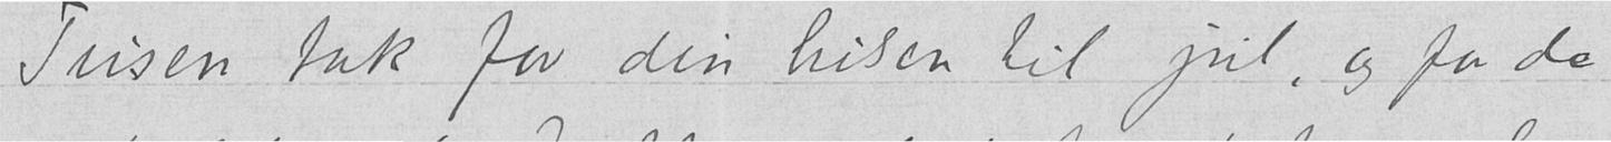Tusen tak for din hilsen til jul, og for de
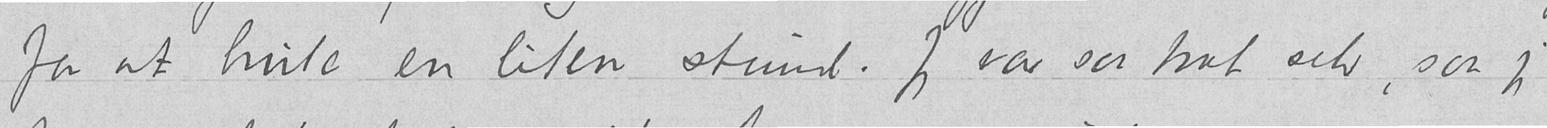for at hvile en liten stund. Jeg var saa træt selv, saa jeg
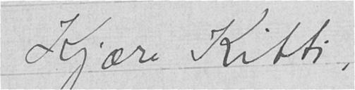Kjære Kitti,
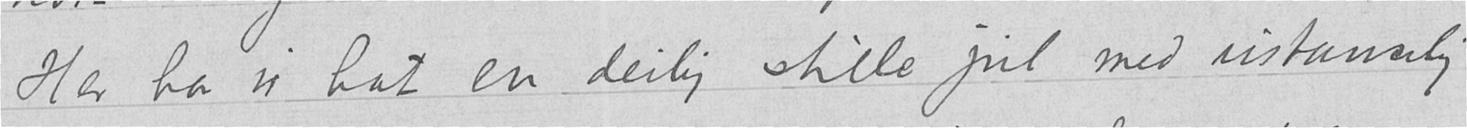Her har vi hat en deilig stille jul med ustanselig
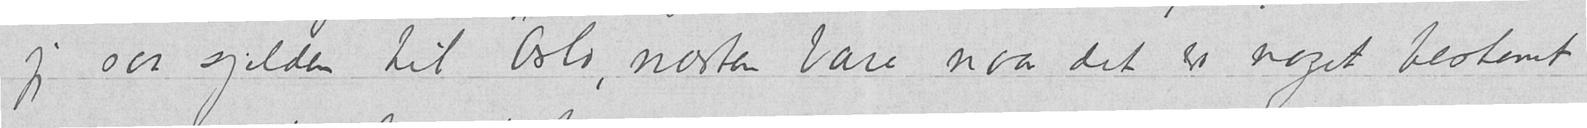jeg saa sjelden til Oslo, næsten bare naar det er noget bestemt
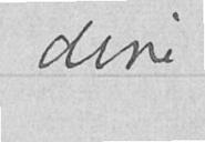dine
